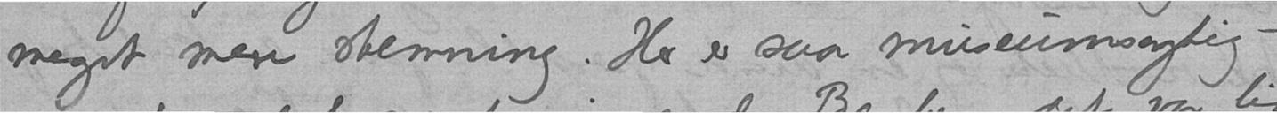meget mere stemning. Her er saa museumsagtig -
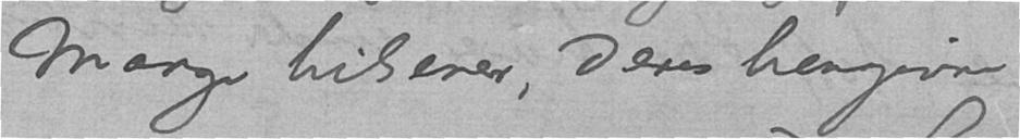Mange hilsener, Deres hengivne
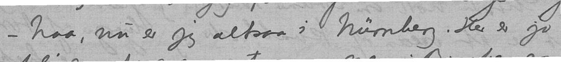- Naa, nu er jeg altsaa i Nürnberg. Her er jo
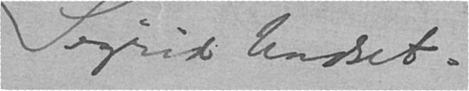Sigrid Undset.
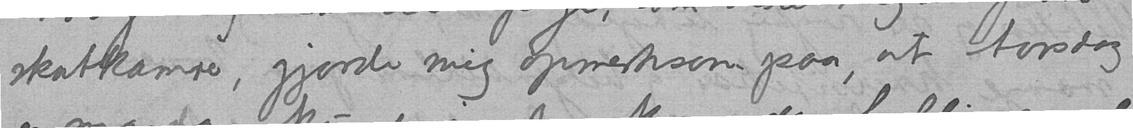skatkamre, gjorde mig opmerksom paa at torsdag
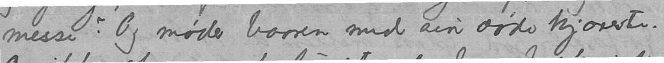messe? Og møder baaren med sin døde kjæreste.
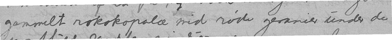gammelt rokokopalæ med røde geranier under de
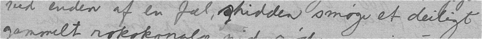ved enden af en fæl, skidden smøge et deiligt
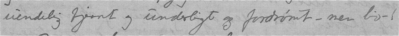uendelig fjernt og underligt og fordrømt - men liv -!
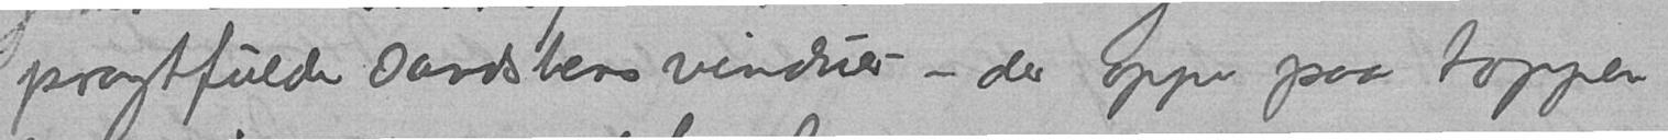pragtfulde sandstens vinduer - der oppe paa toppen
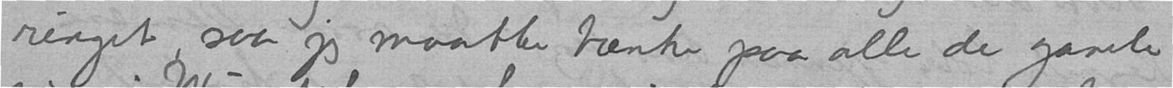ringet, saa jeg maatte tænke paa alle de gamle
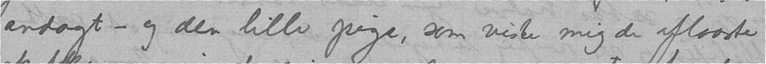andagt - og den lille pige, som viste mig de aflaaste
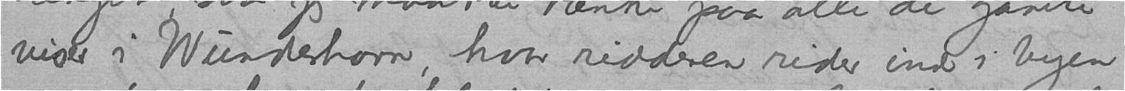viser i Wunderhaven, hvor ridderen rider ind i byen
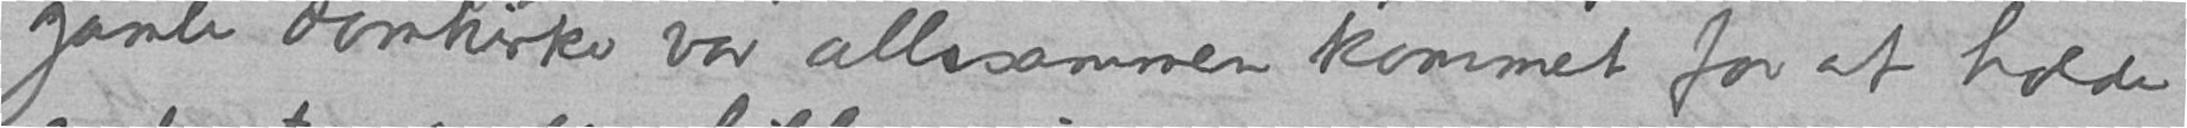gamle domkirke var allesammen kommet for at holde
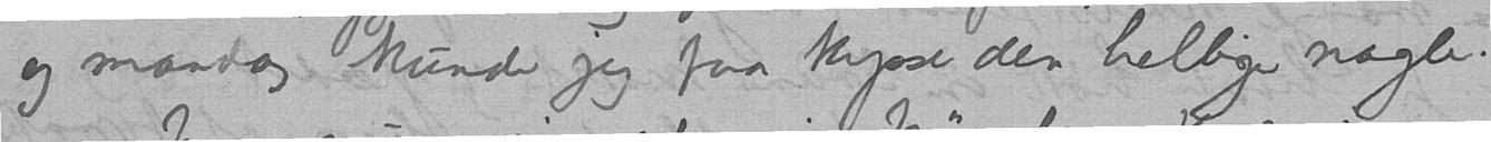og mandag kunde jeg faa kysse den hellige nagle.
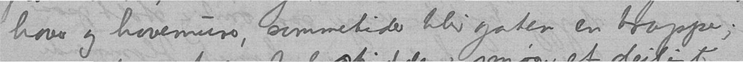haver og havemure, sommetider blir gaten en trappe;
